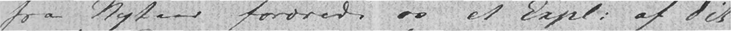fra Nytaar forærede os et Expl: af dit
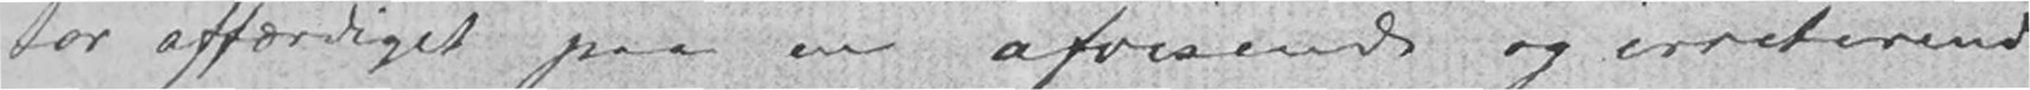tor affærdiget paa en afvisende og irriterende
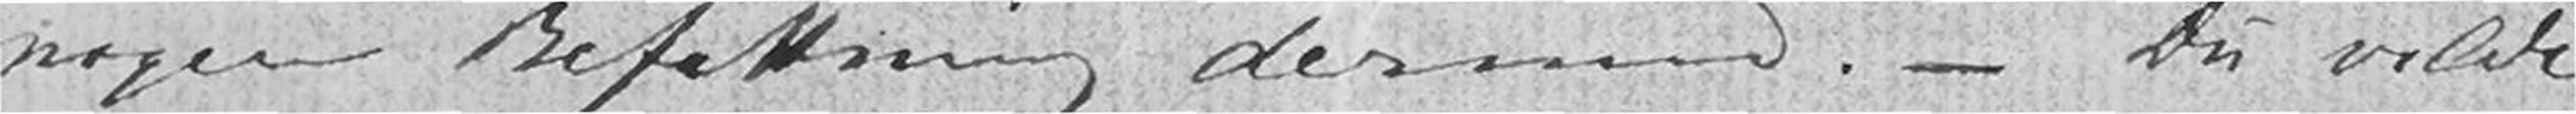nogen Befattning dermed.- Du vilde
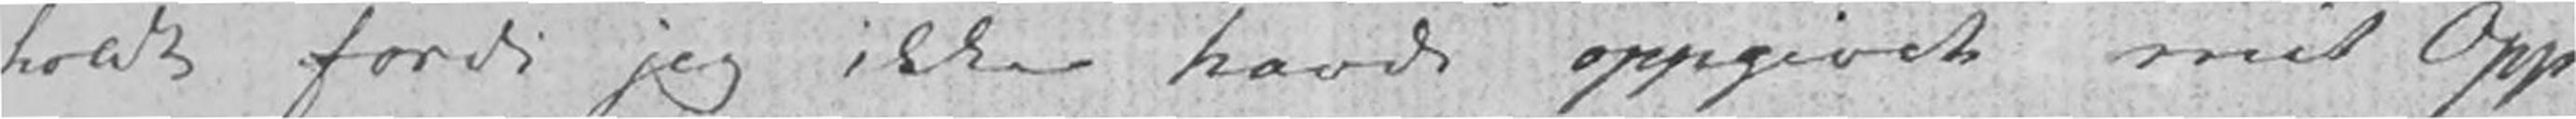holdt fordi jeg ikke havde oppgivet mit Opp-
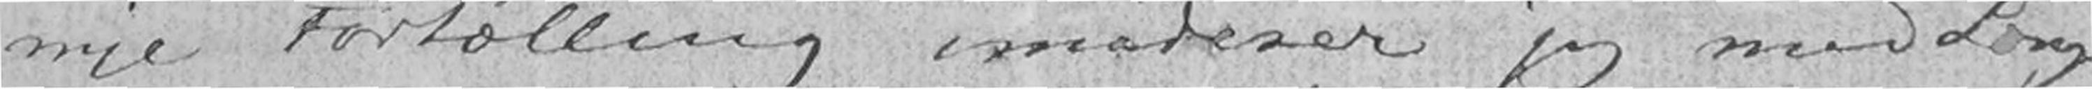nye Fortælling imødeser jeg med Læng-
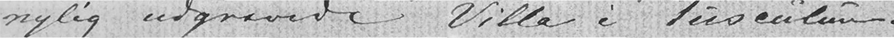nylig udgravede Villa i Tusculum.
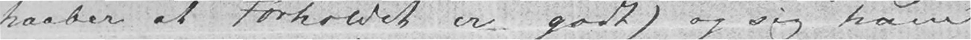haaber at Forholdet er godt) og sig ham
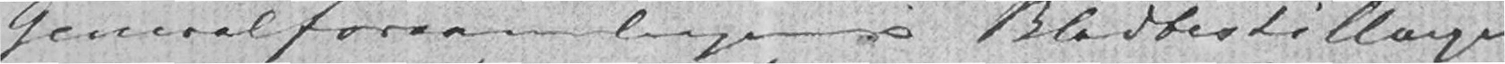Generalforsamlingens Bladbestillinger
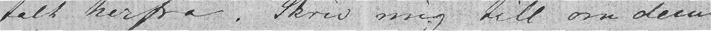talt herfra. Skriv mig till om denne
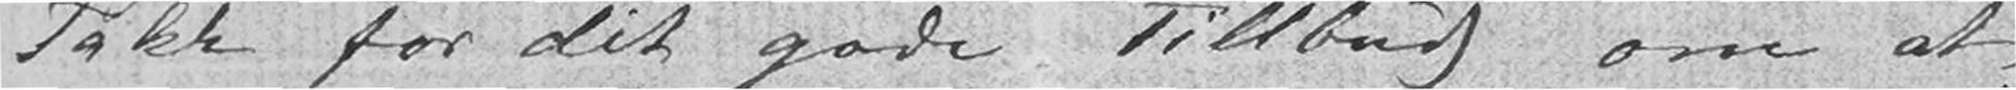Takk for dit gode Tillbud om at
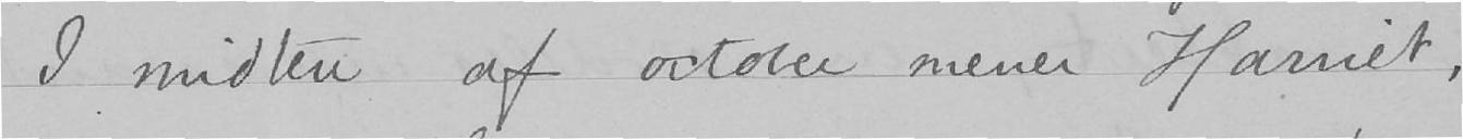I midten af october mener Harriet,
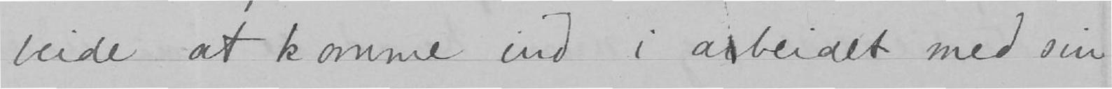beide at komme ind i arbeidet med sin
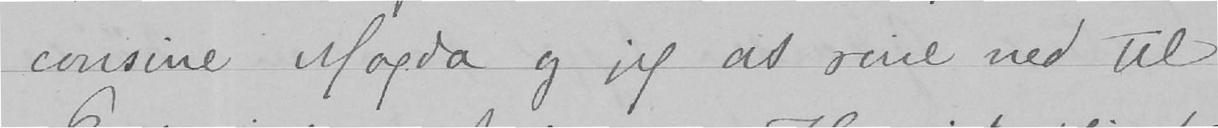cousine Magda og jeg at reise ned til
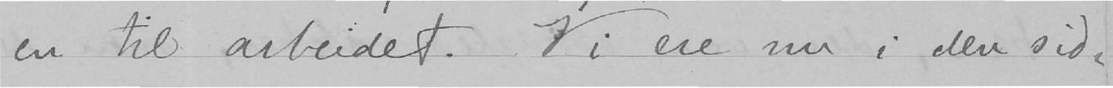en til arbeidet. Vi ere nu i den sid-
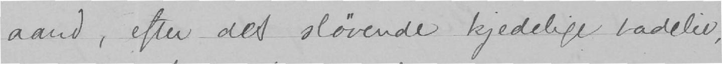aand, efter det sløvende kjedelige badeliv,
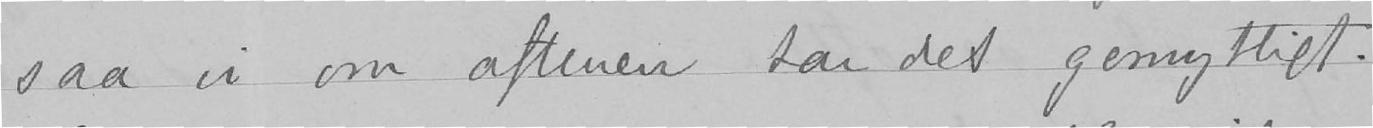saa vi om aftenen har det gemytligt.
